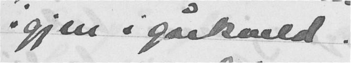igjen i gårkveld.
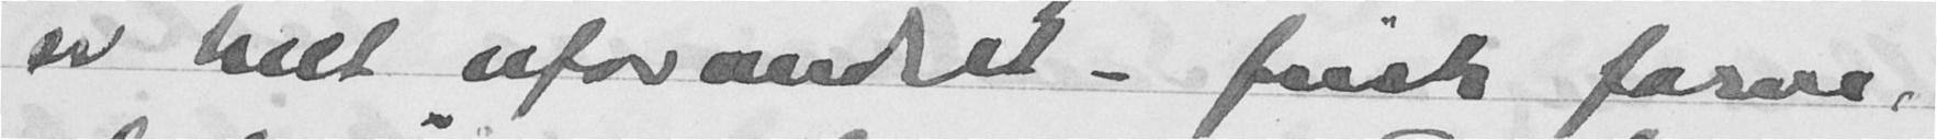er helt uforandret - frisk farve,
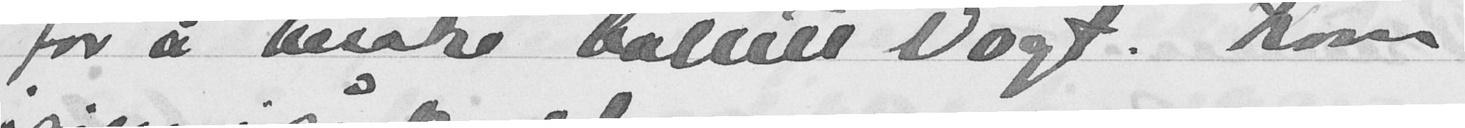for å besøke Kollett Vogt. Kom
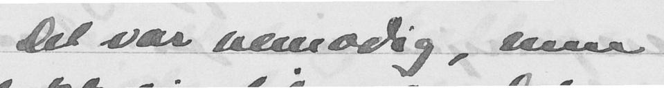Det var vemodig, men
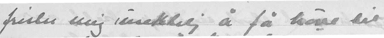frister mig unektelig å få have til
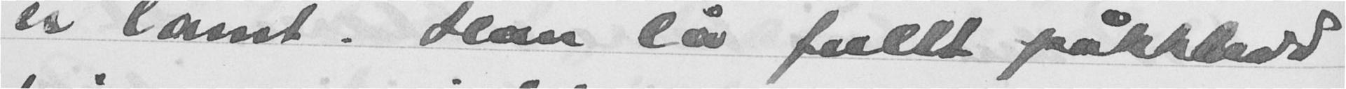er lamt. Han lå fullt påkledd
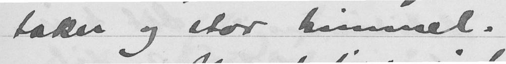taker og stor himmel.
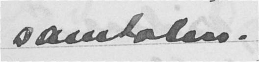samtalen.
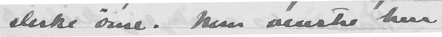sterke øine. Men venstre kne
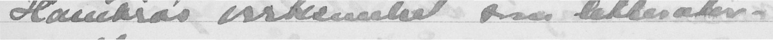Hambro's virksomhet som litteratur-
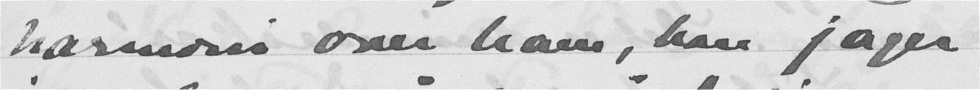harmoni over ham, han jager
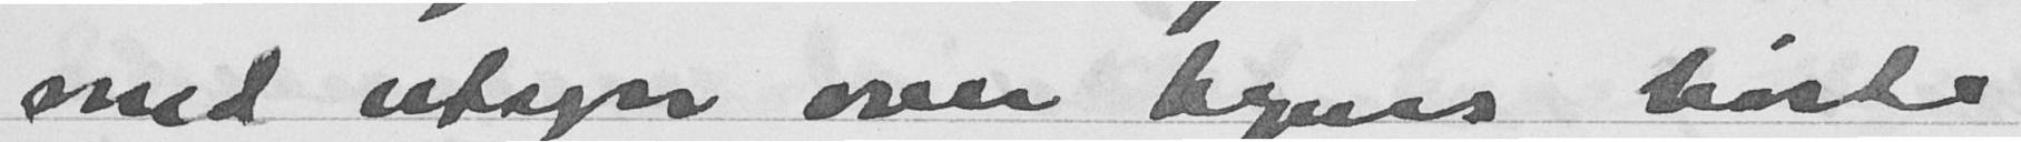med utsyn over byens hvite
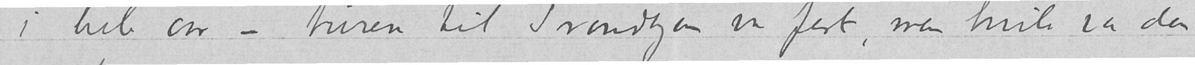i hele aar – turen til Trondhjem var fest, men hvile var den
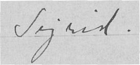Sigrid.
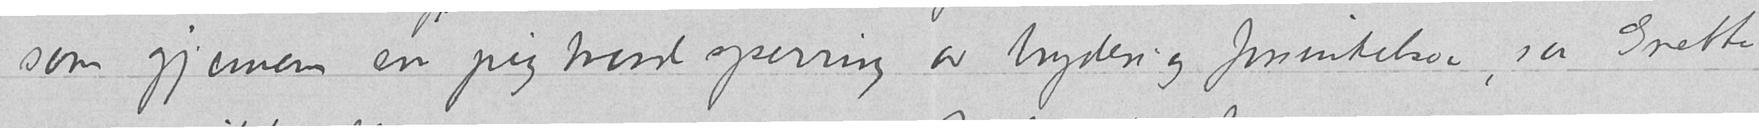som gjennem en pigtraadsperring av bryderi og forsinkelser, saa Grette
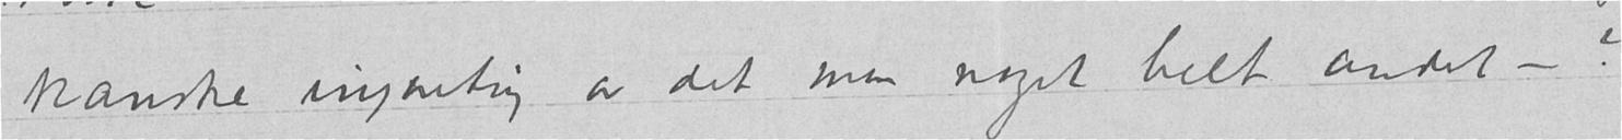kanske ingenting av det men noget helt andet –?
